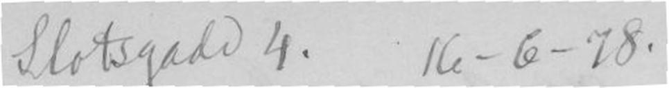Slotsgade 4. 16-6-78.
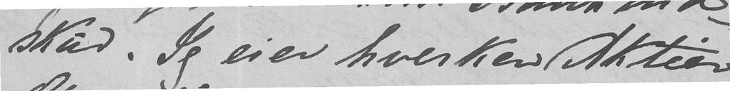skud. Jeg eier hverken Aktier
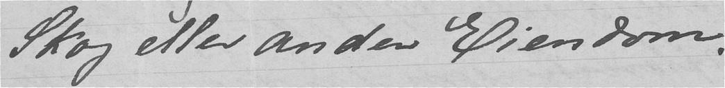Skog eller anden Eiendom.
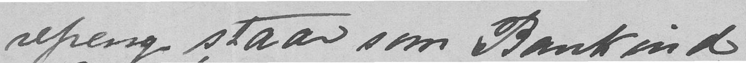repeng staar som Bankind-
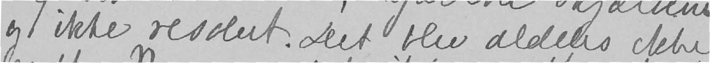og ikke resolut. Det blev aldeles ikke
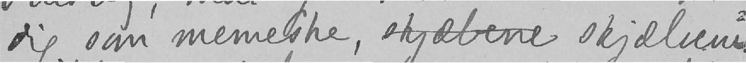dig som menneske, skjæbene skjælvenes
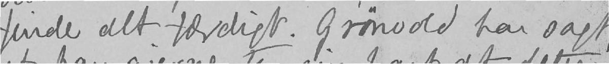finde alt færdigt. Grønvold har sagt,
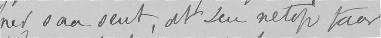ned saa sent, at Du netop faar
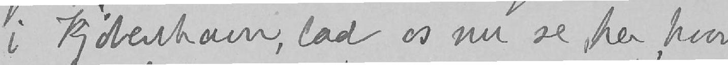i Kjøbenhavn, lad os nu se her, hvor
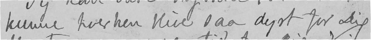kunne hverken blive saa dyrt for Dig
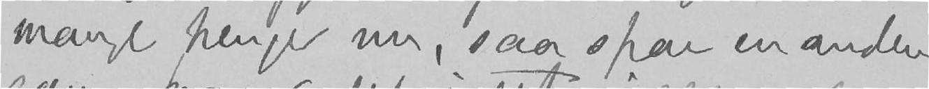mange penge nu, saa spar en anden
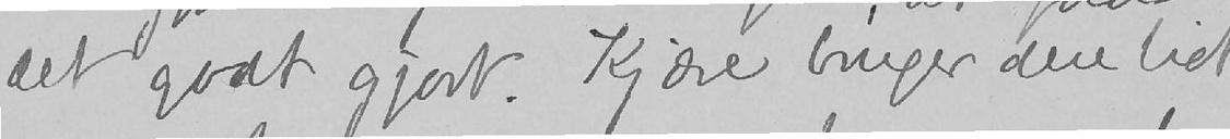det godt gjort. Kjære bruger dere lidt
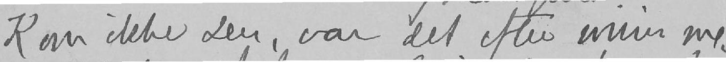Kan ikke Du, var det efter min me-
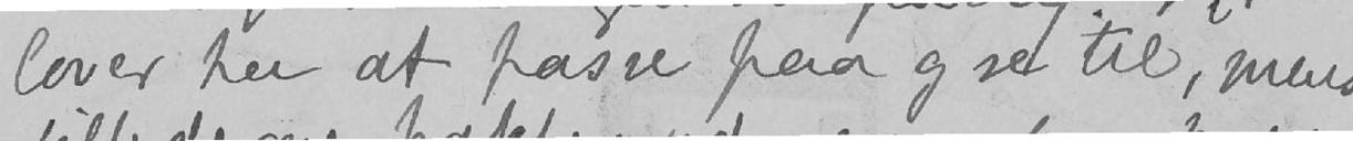lover her at passe paa og se til, mens
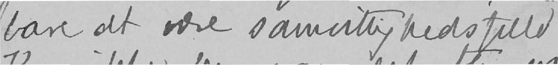bare at være samvittighedsfuld.
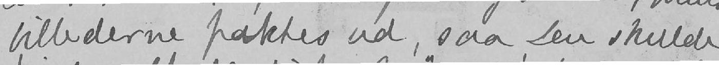billederne fraktes ud, saa Du skulde
